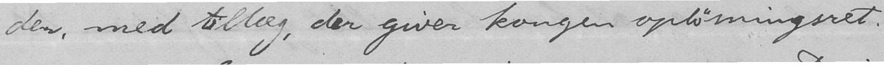der, med tillæg, der giver kongen opløsningsret.
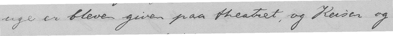uge er bleven given paa theatret, og Keiser og
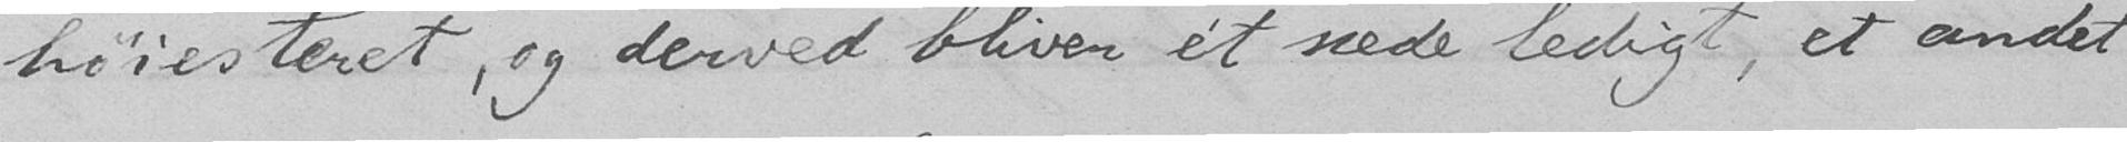høiesteret, og derved bliver et sæde ledigt, et andet
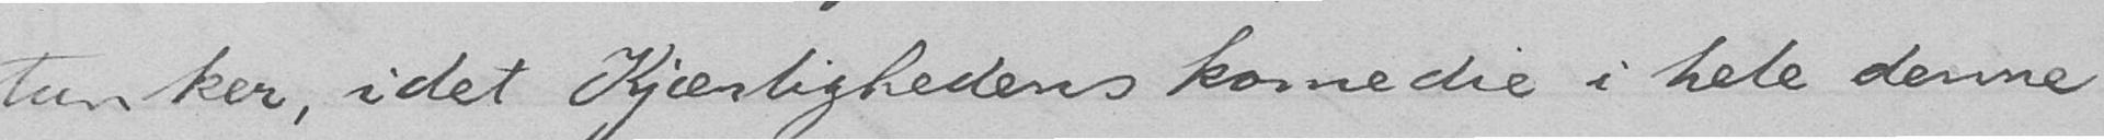tanker, idet Kjærlighedens komedie i hele denne
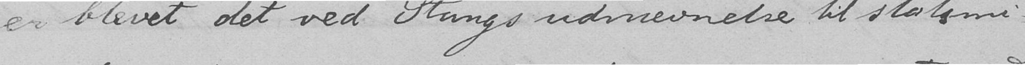er blevet det ved Stangs udnævnelse til statsmi-
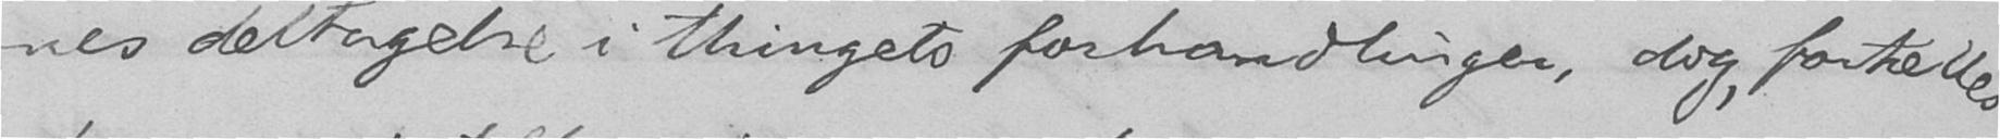nes deltagelse i thingets forhandlinger, dog, fortælles
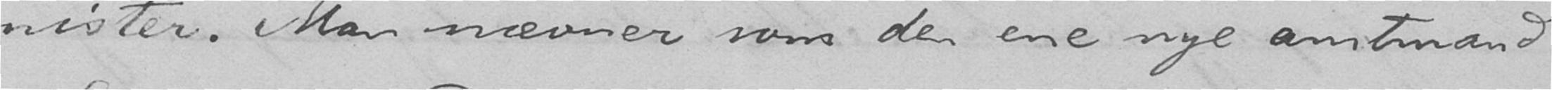nister. Man nævner som den ene nye amtmand
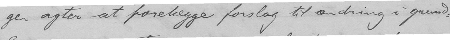gen agter at forelægge forslag til ændring i grund-
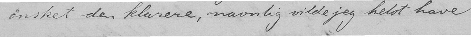ønsket den klarere, navnlig vilde jeg helst have
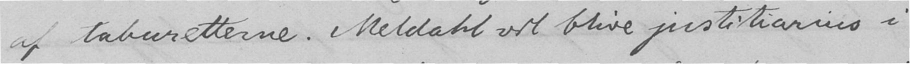af taburetterne. Meldahl vil blive justitiarius i
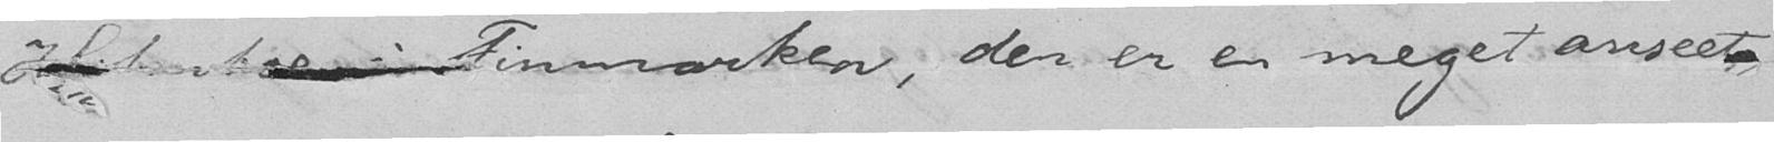Finmarken, der er en meget anseet
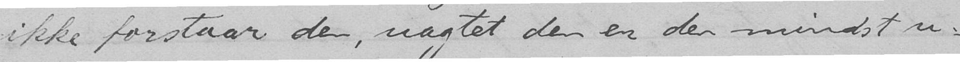ikke forstaar den, uagtet den er den mindst u-
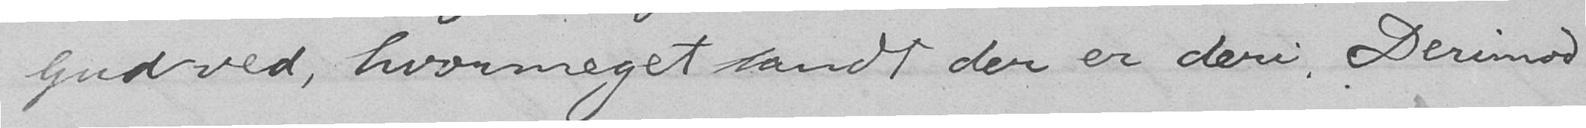Gud ved, hvormeget sandt der er deri. Derimod
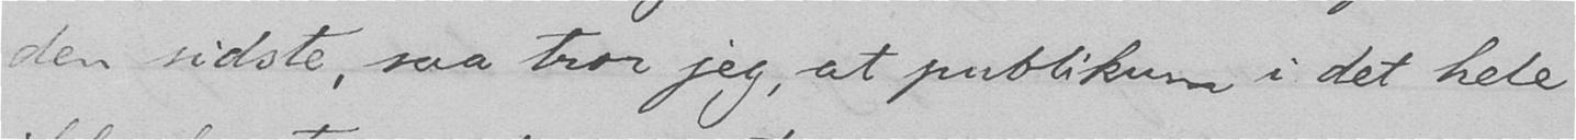den sidste, saa tror jeg, at publikum i det hele
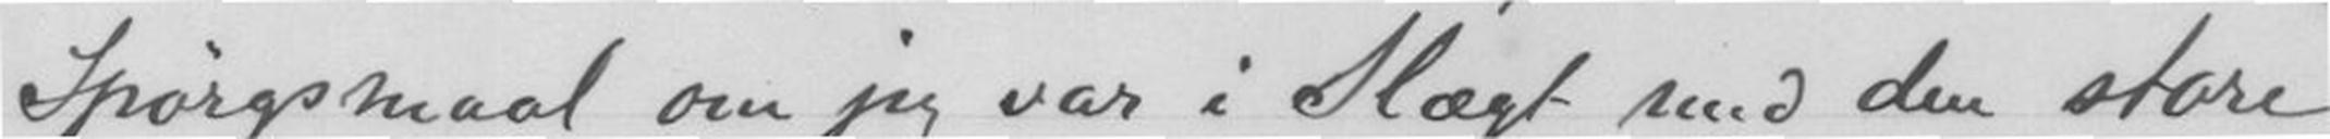Spørgsmaal om jeg var i Slægt med den store
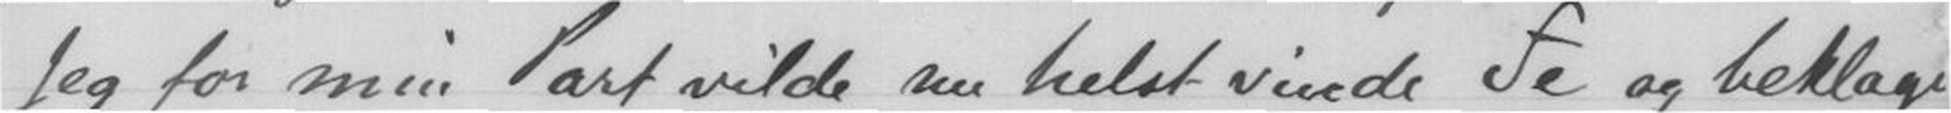Jeg for min Part vilde nu helst vinde Fe og beklage
